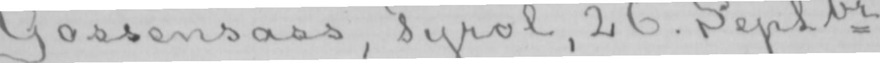Gossensass, Tyrol, 26. Septbr
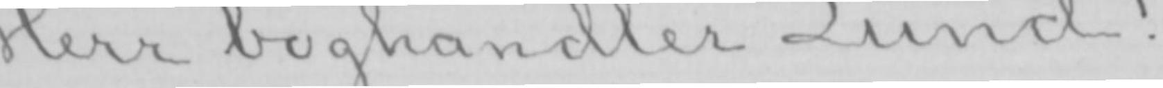Herr boghandler Lund!
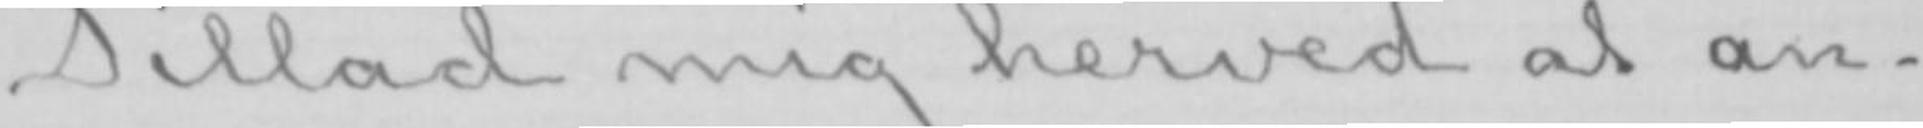Tillad mig herved at an-
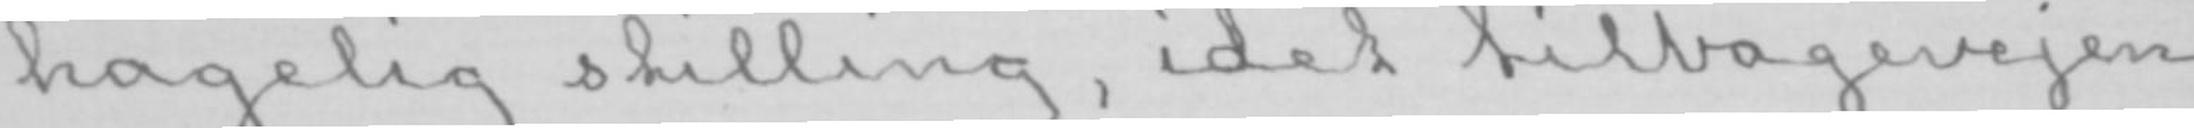hagelig stilling, idet tilbagevejen
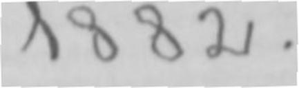1882.
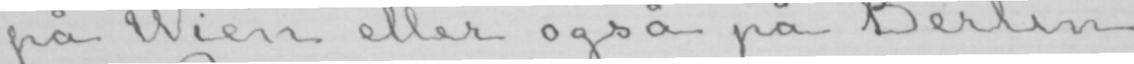på Wien eller også på Berlin
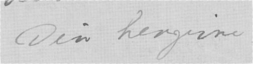Din hengivne
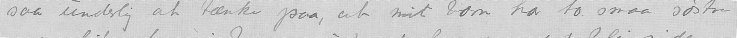saa underlig at tænke paa, at mit barn har to smaa søstre
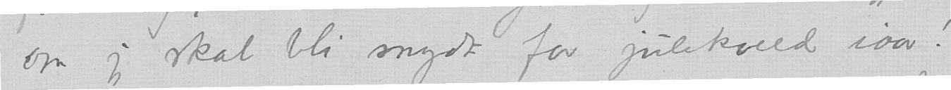om jeg skal bli snydt for julekveld iaar!
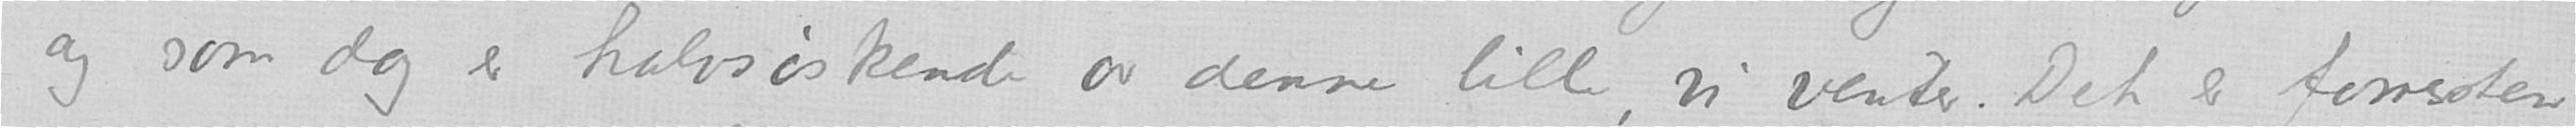og som dog er halvsøskende av denne lille, vi venter. Det er forresten
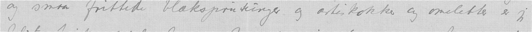og smaa frittede blæksprutunger og artiskokker og omeletter er jeg
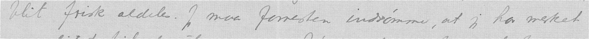blit frisk aldeles. Jeg maa forresten indrømme, at jeg har merket
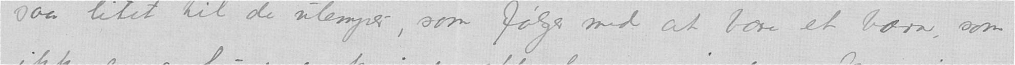saa litet til de ulemper, som følger med at bære et barn, som
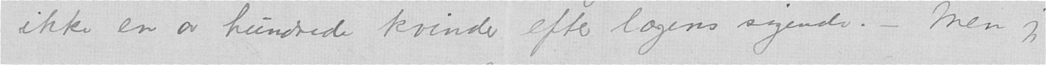ikke en av hundrede kvinder efter lægens sigende. - Men jeg
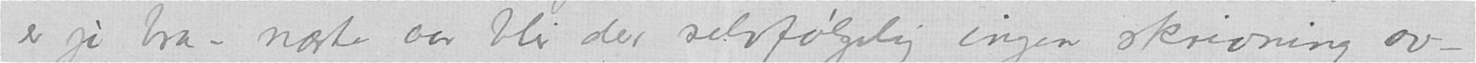er jo bra - næste aar blir der selvfølgelig ingen skrivning av -.
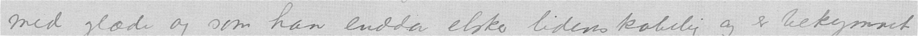med glæde og som han endda elsker lidenskabelig og er bekymret
